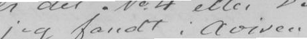jeg fandt i Avisen
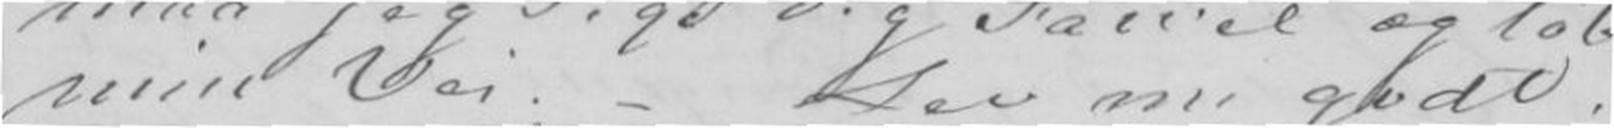min Vei. -Lev nu godt,
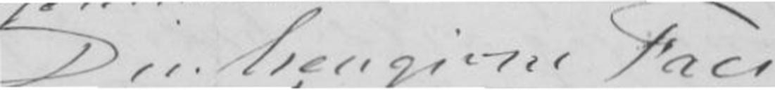Din hengivne Faer
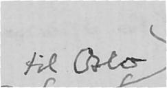til Oslo
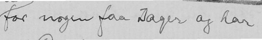for nogen faa Dager og har
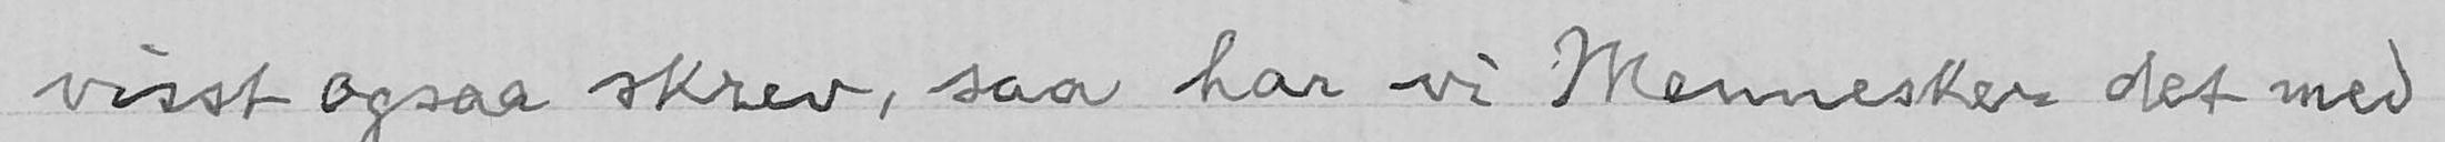visst ogsaa skrev, saa har vi Mennesker det med
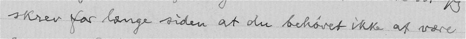skrev for længe siden at du behøvet ikke at være
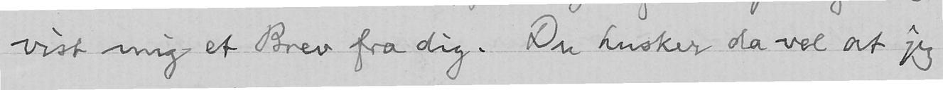vist mig et Brev fra dig. Du husker da vel at jeg
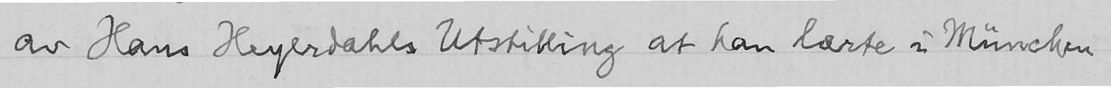av Hans Heyerdahls Utstilling at han lærte i München
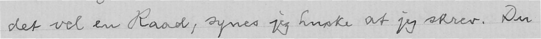det vel en Raad, synes jeg huske at jeg skrev. Du
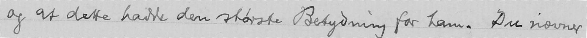og at dette hadde den største Betydning for ham. Du nævner
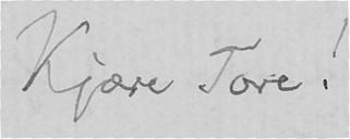Kjære Tore!
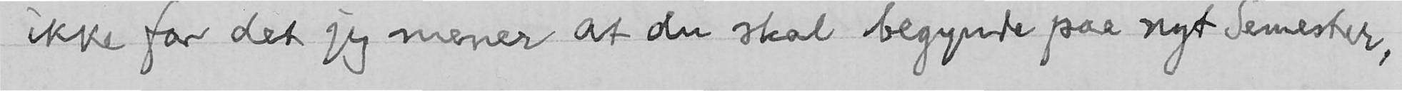ikke for det jeg mener at du skal begynde paa nyt Semester,
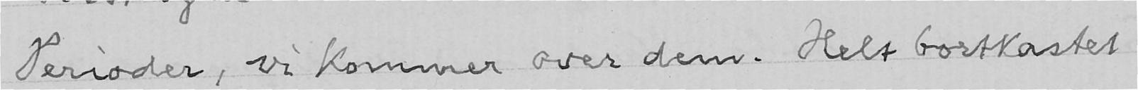Perioder, vi kommer over dem. Helt bortkastet
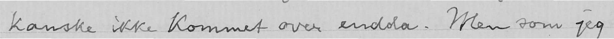kanske ikke kommet over endda. Men som jeg
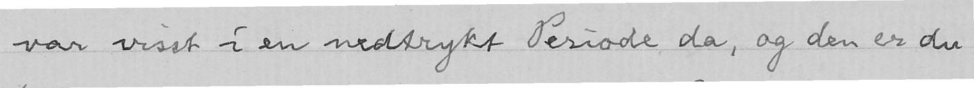var visst i en nedtrykt Periode da, og den er du
